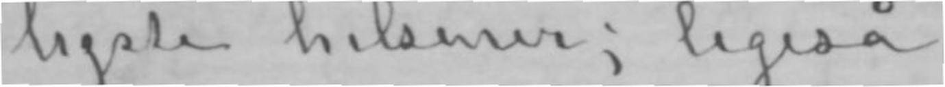ligste hilsener; ligeså
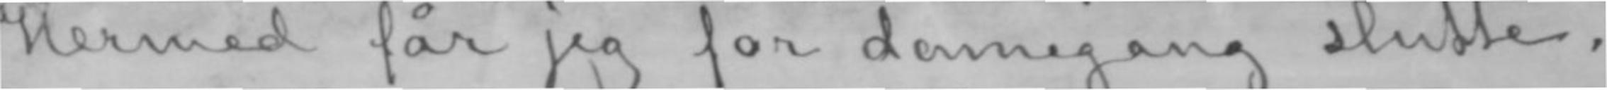Hermed får jeg for dennegang slutte.
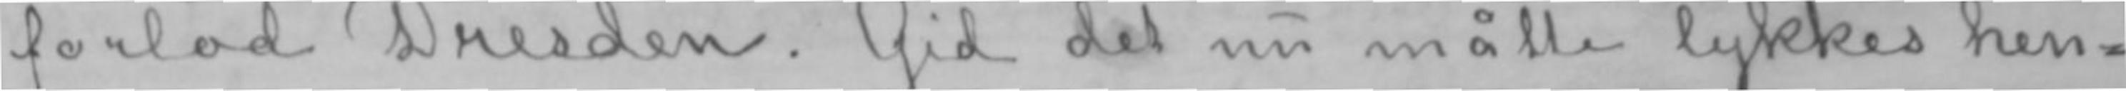forlod Dresden. Gid det nu måtte lykkes hen-
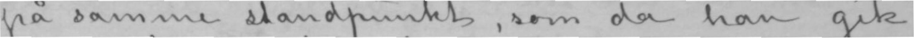på samme standpunkt, som da han gik
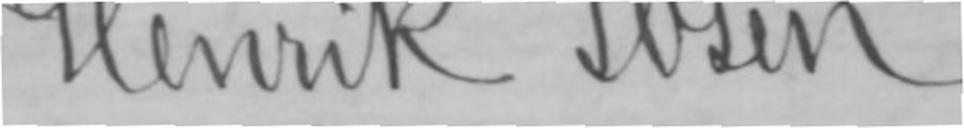Henrik Ibsen.
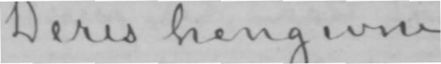Deres hengivne
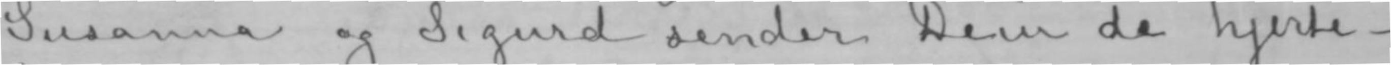Susanna og Sigurd sender Dem de hjerte-
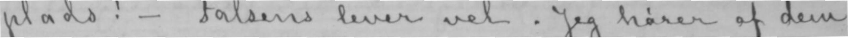plads!- Falsens lever vel. Jeg hører af dem
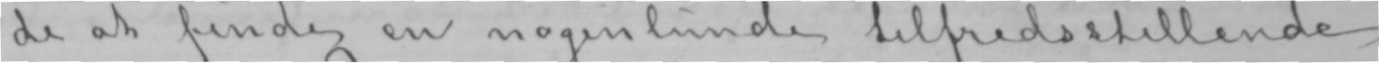de at finde en nogenlunde tilfredsstillende
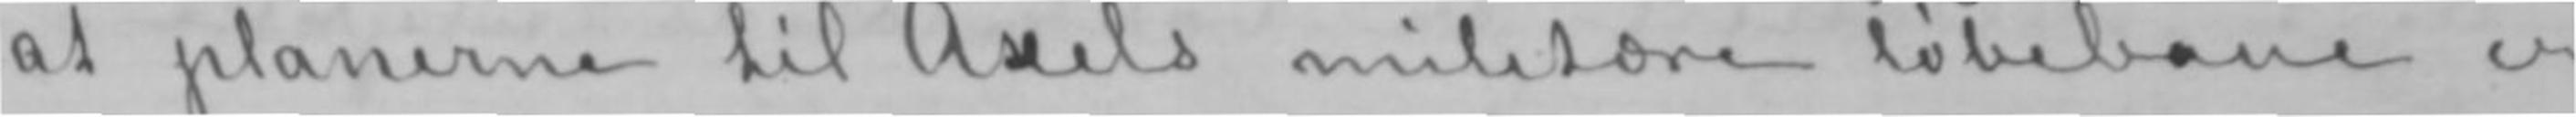at planerne til Axels militære løbebane er
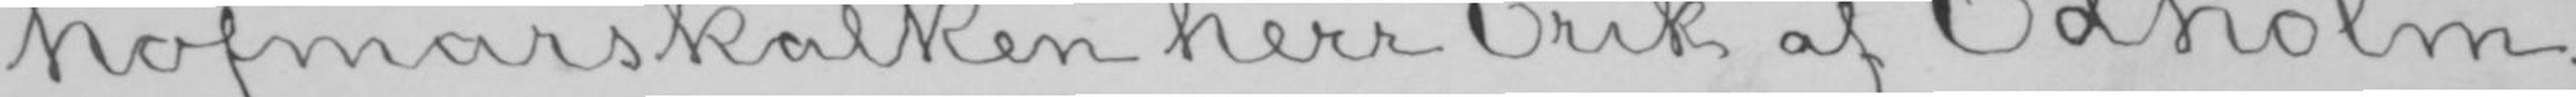hofmarskalken herr Erik af Edholm.
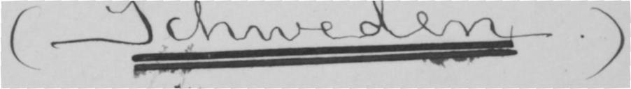(Schweden.)
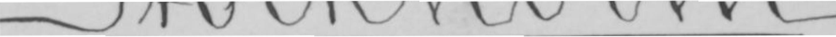Stockholm.
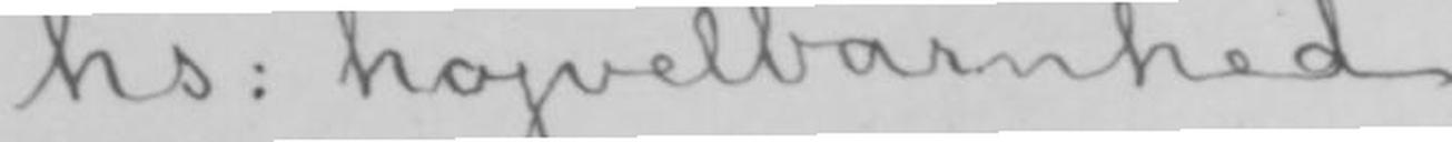hs: højvelbårnhed
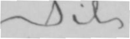Til
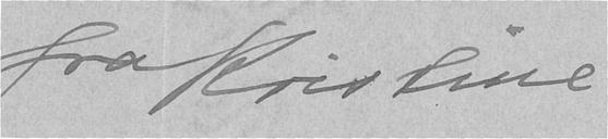fra Kristine
